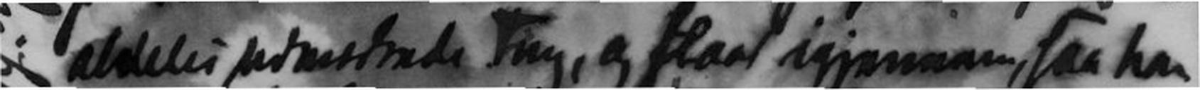aldeles udmærkede Ting, og slaat igjennem, saa han
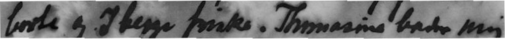borte og I begge friske. Thomasine beder mig
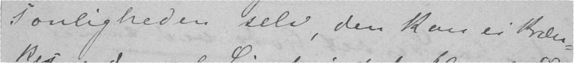sonligheden selv, den kan ei kræn-
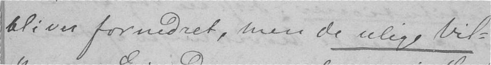bliver fornedret, men de ulige Vil-
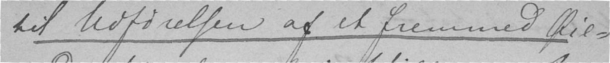til Udførelsen af et fremmed Øie-
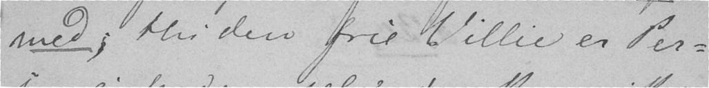med; thi den frie Villie er Per-
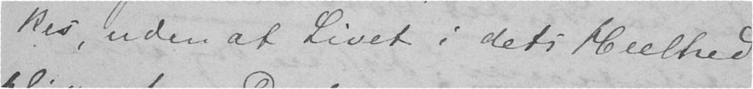kes, uden at Livet i dets Heelhed
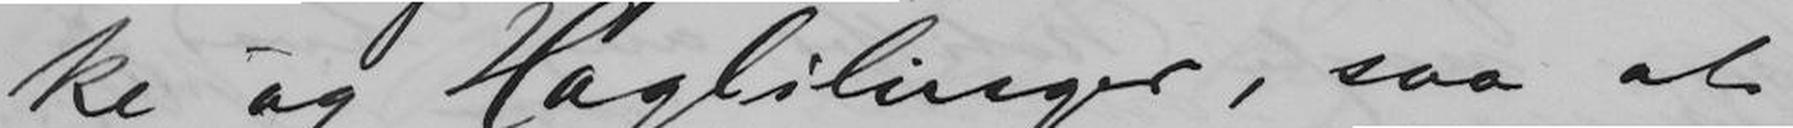ke og Haglilinger, saa at
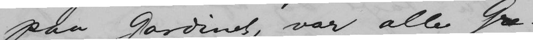paa Gardinet, var alle Gre-
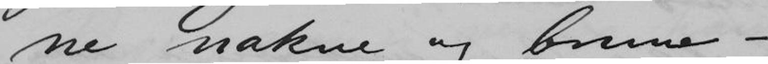ne nakne og brune - og
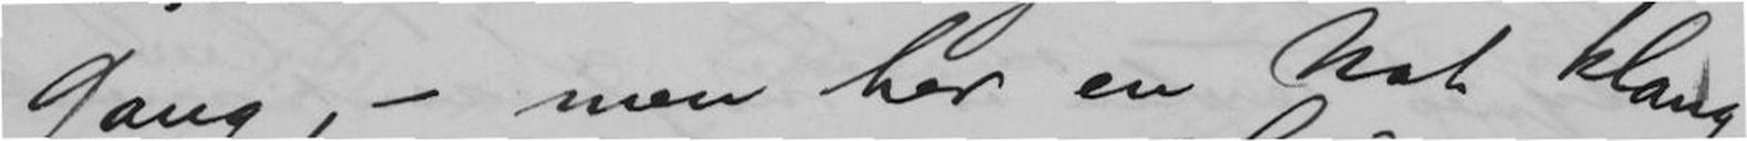Gang, - men her en Nat klang
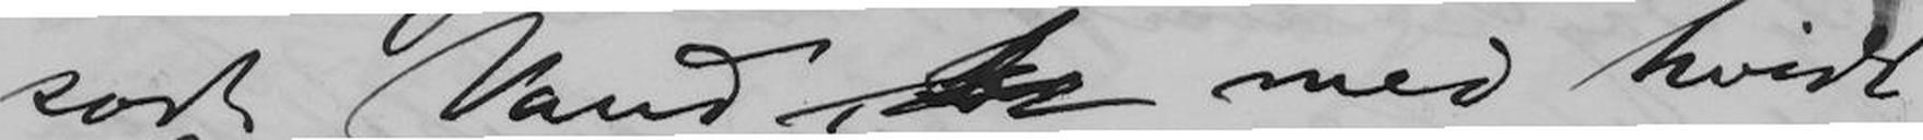sort Vand med hvidt
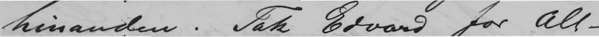hinanden. Tak Edvard for Alt-
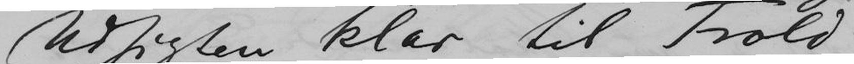Udsigten klar til Trold-
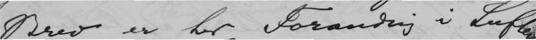Brev er her Forandrig i Luften.
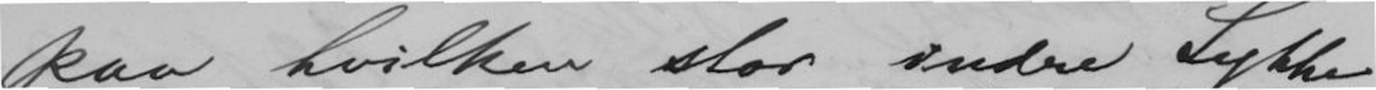paa hvilken stor indre Lykke
